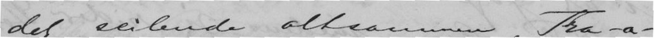det seilende altsammen, Tra-a-
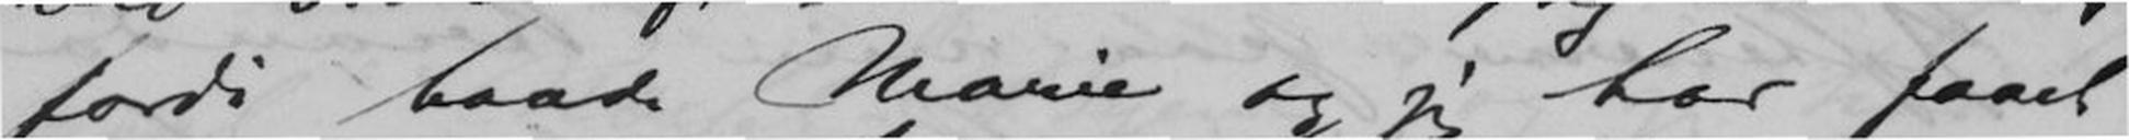fordi baade Marie og jeg har faaet
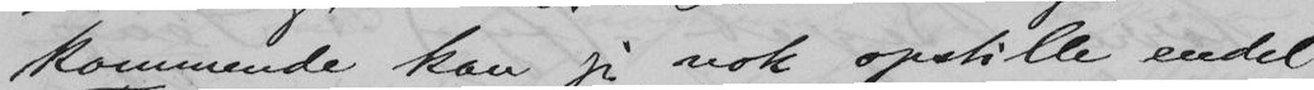kommende kan jeg nok opstille endel
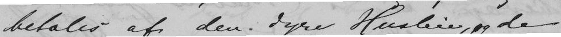betales af den dyre Husleie, og de
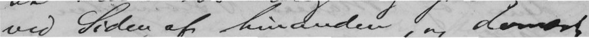ved Siden af hinanden, og dernæst
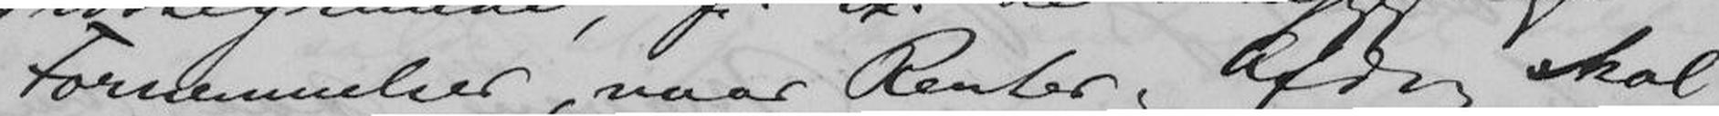Fornemmelser, naar Renter og Afdrag skal
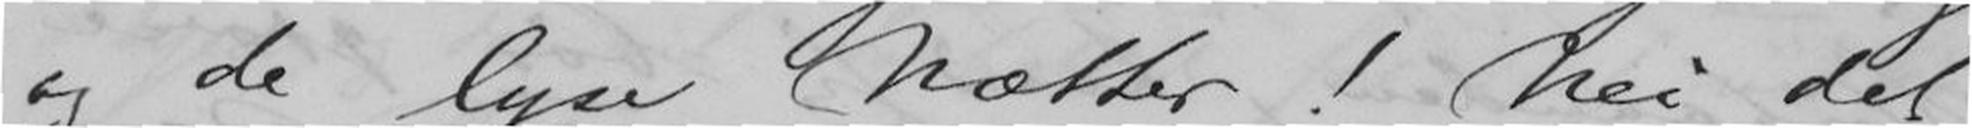og de lyse Nætter! Nei det
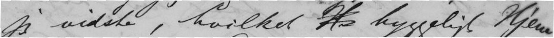jeg vidste, hvilket H hyggeligt Hjem,
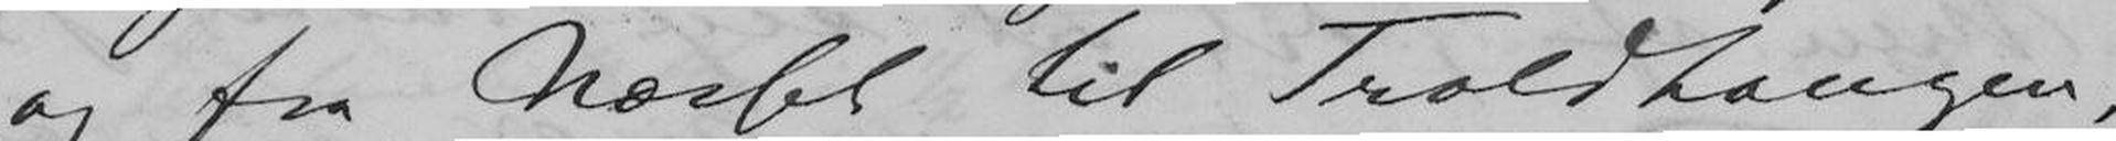og fra Næsset til Troldhaugen,
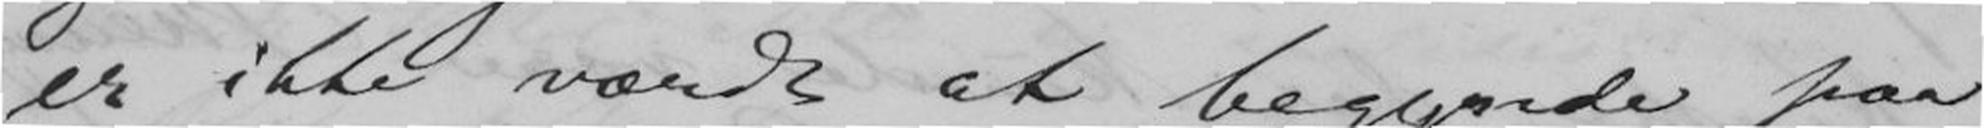er ikke værdt at begynde paa
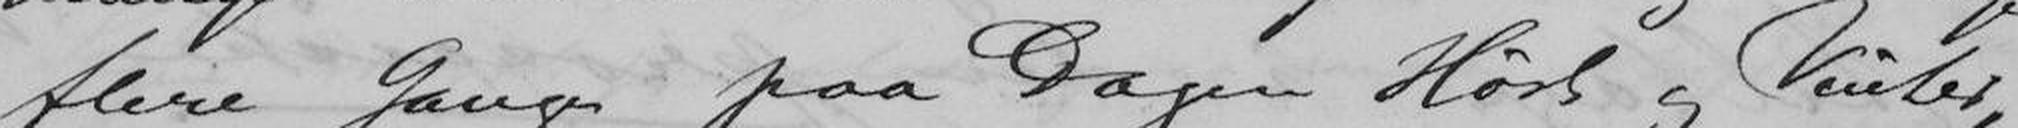flere Gange paa Dagen Høst og Vinter,
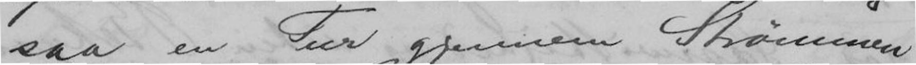saa en Tur gjennem Strømmen
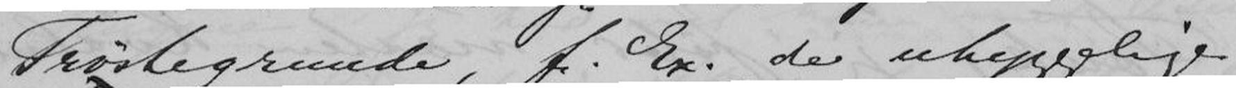Trøstegrunde, f. Ex. de uhyggelige
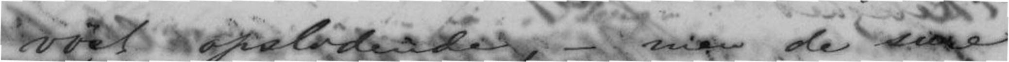vøst opslidende, - men de sure
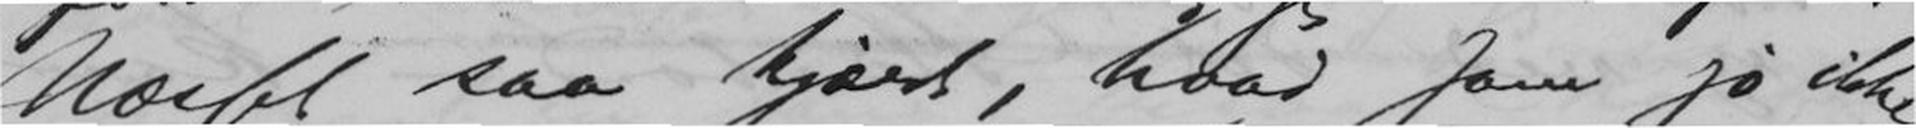Næsset saa kjært, hvad som jo ikke
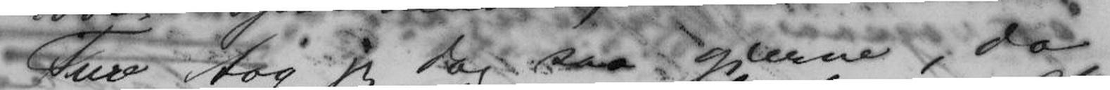Ture tog jeg dog saa gjerne, da
